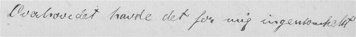Overhovedet havde det for mig ingensomhelst
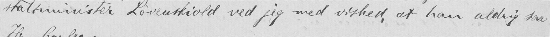statsminister Løvenskiold ved jeg med vished, at han aldrig saa
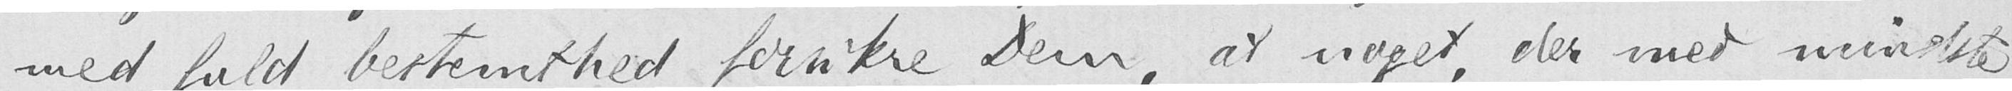med fuld bestemthed forsikre Dem, at noget, der med mindste
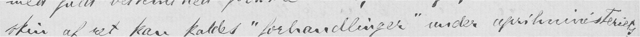skin af ret kan kaldes "forhandlinger" under aprilministeriets
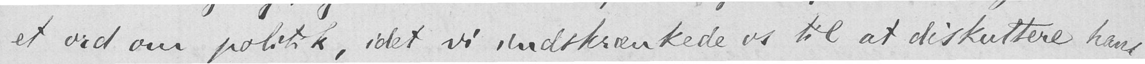et ord om politik, idet vi indskrænkede os til at diskuttere hans
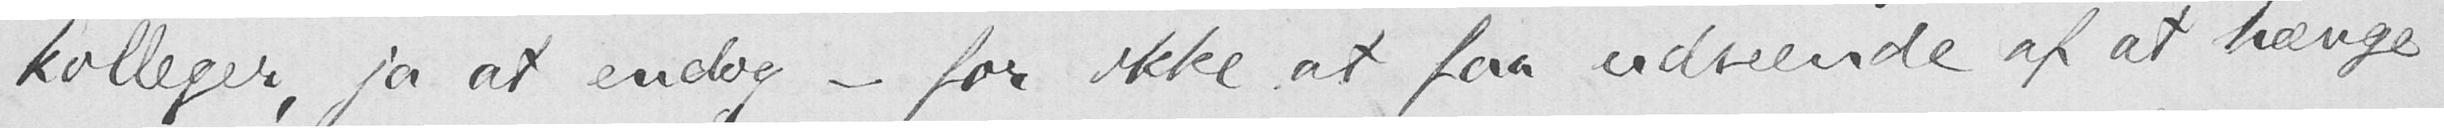kolleger, ja at endog - for ikke at faa udseende af at hænge
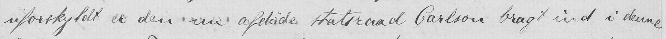uforskyldt er den nu afdøde statsraad Carlson bragt ind i denne
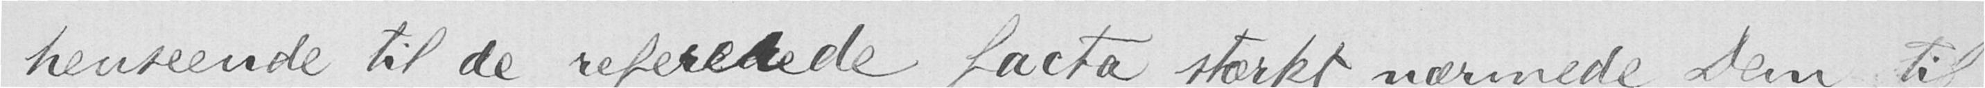henseende til de refererede facta stærkt nærmede Dem til
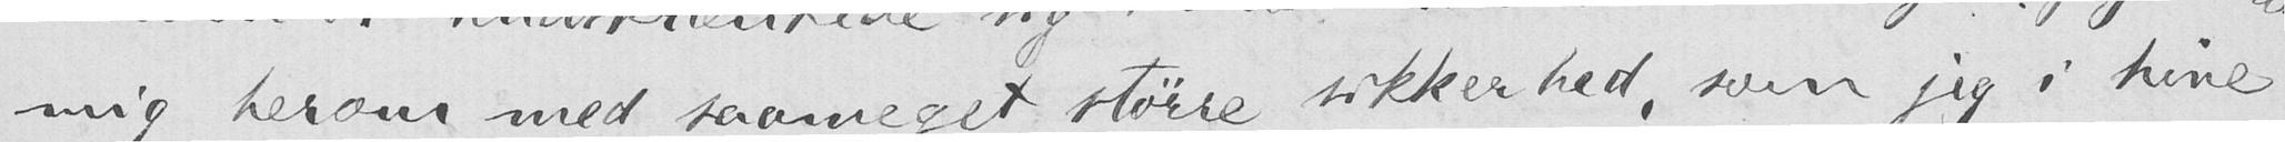mig herom med saameget større sikkerhed, som jeg i hine
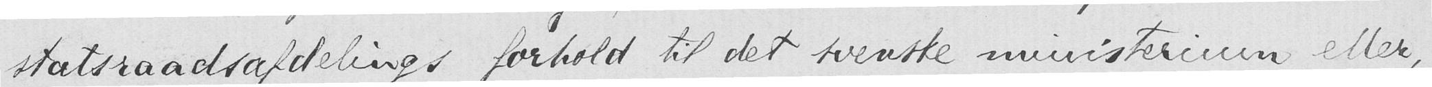statsraadsafdelings forhold til det svenske ministerium eller,
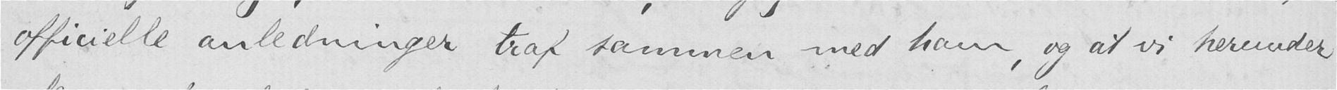officielle anledninger traf sammen med ham, og at vi herunder
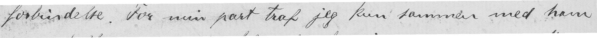forbindelse. For min part traf jeg kun sammen med ham
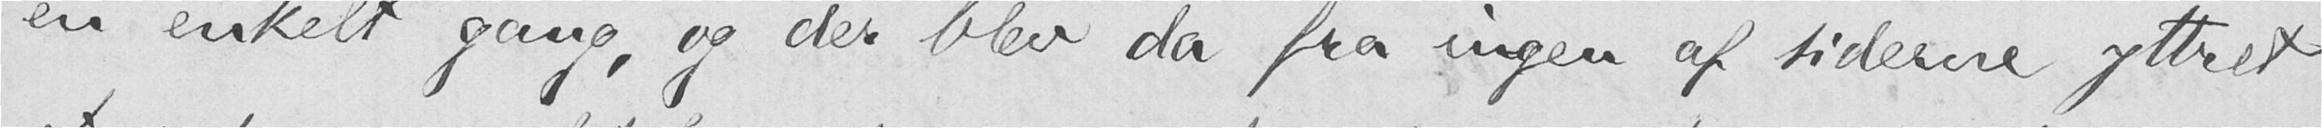en enkelt gang, og der blev da fra ingen af siderne yttret
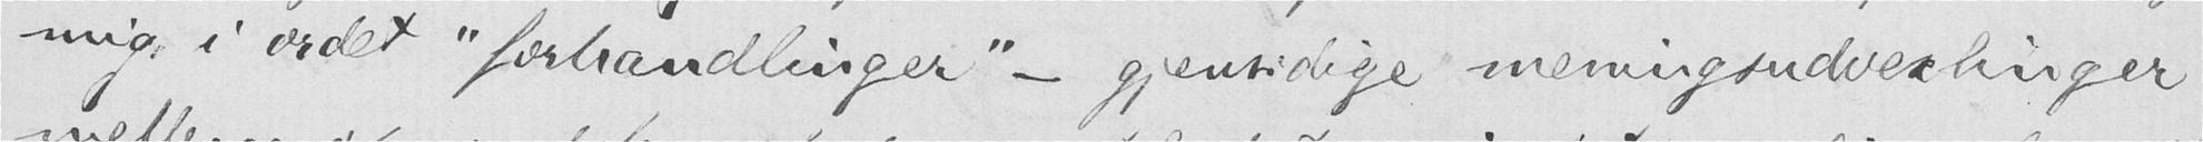mig i ordet "forhandlinger" - gjensidige meningsudvexlinger
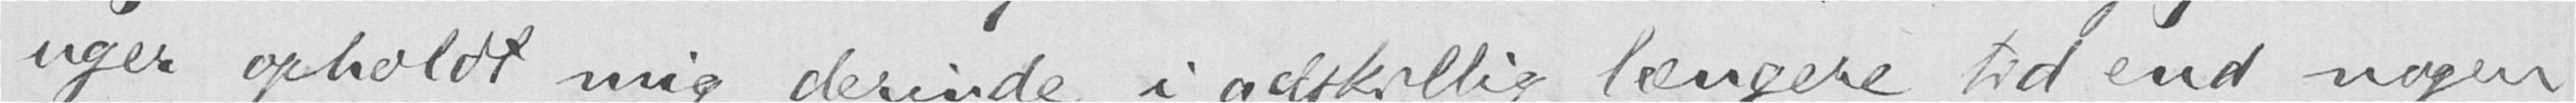uger opholdt mig derinde i adskillig længere tid end nogen
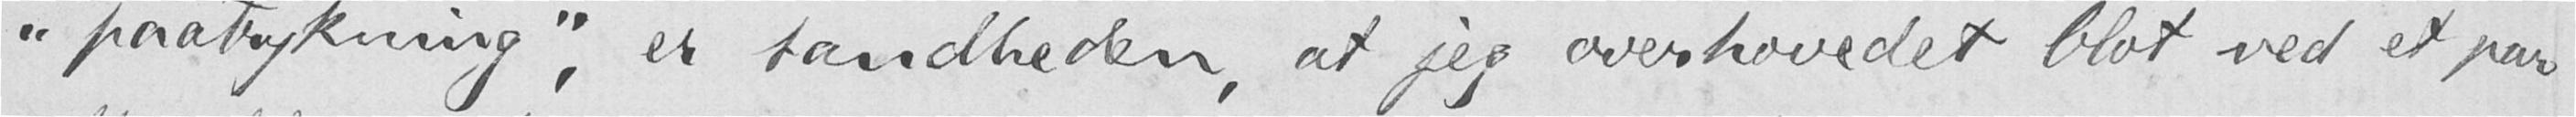"paatrykning", er sandheden, at jeg overhovedet blot ved et par
